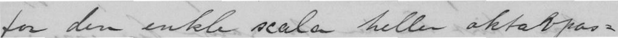for den enkle scala heller oktavpas-
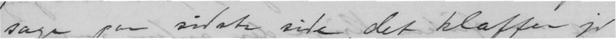sage paa sidste side, det klaffer jo
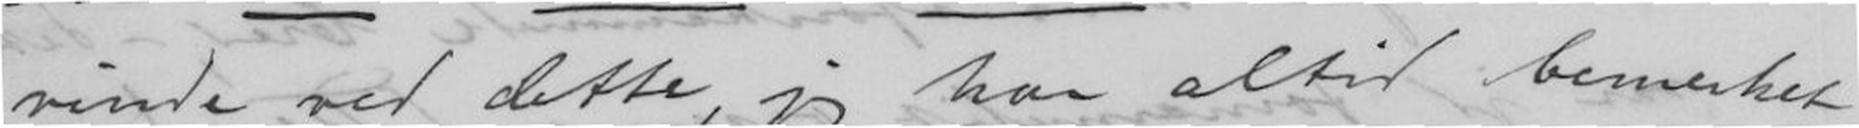vinde ved dette, jeg har altid bemerket
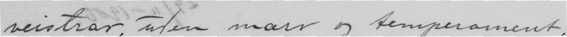veistrav, uden marv og temperament,
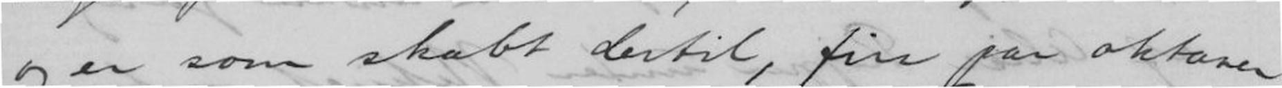og er som skabt dertil, fin paa oktaven
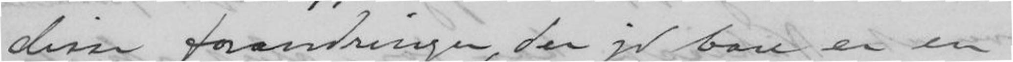disse forandringer, der jo bare er en
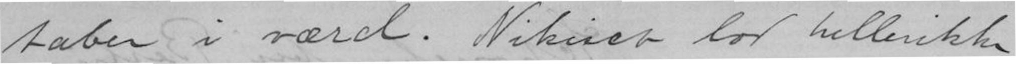taber i værd. Nikisch lod heller ikke
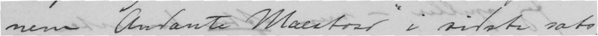nem "Andante Maestoso" i sidste sats,
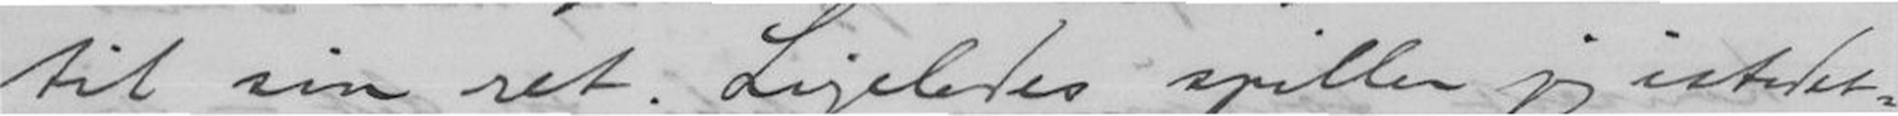til sin ret. Ligeledes spiller jeg istedet-
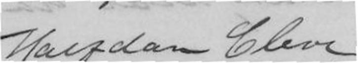Halfdan Cleve.
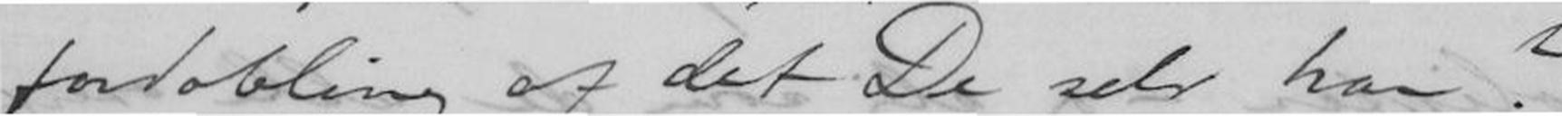fordobling af det De selv har?
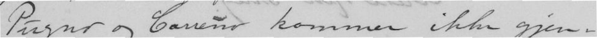Pugno og Carreno kommer ikke gjen-
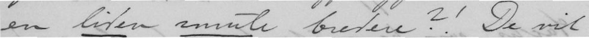en liden smule bredere?! De vil
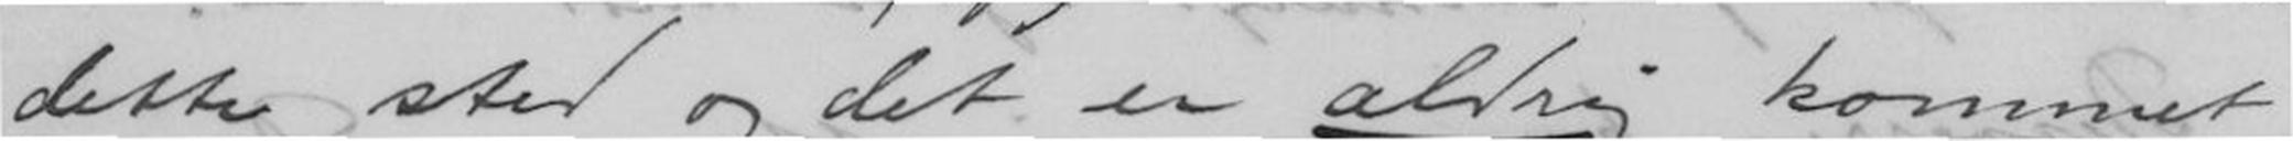dette sted og det er aldrig kommet
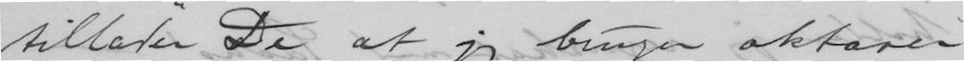tillader De at jeg bruger oktaver,
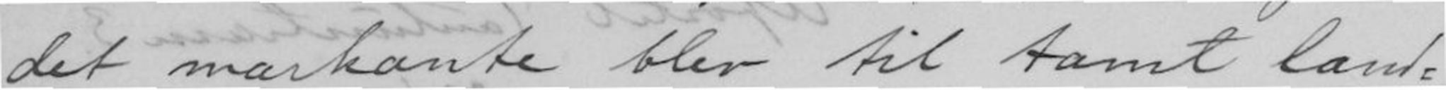det markante blev til tamt land-
